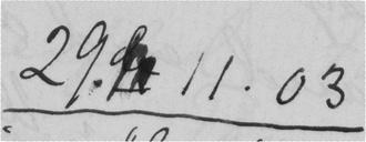29.11.03
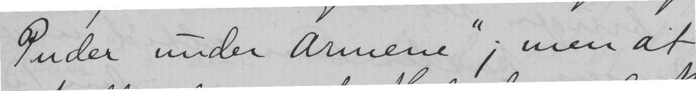Puder under armene"; men at
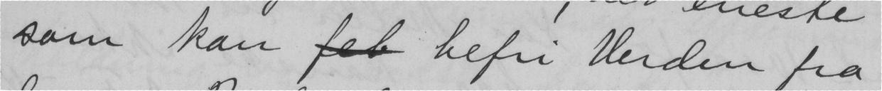som kan feb befri verden fra
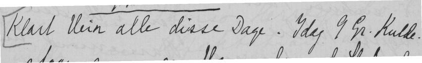Klart veir alle disse Dage. Idag 9 Gr. Kulde.
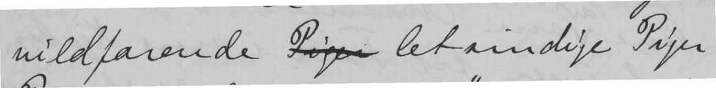vildfarende Piger letsindige Piger
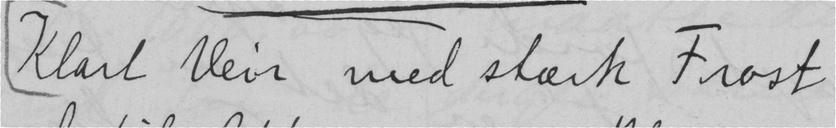Klart veir med stærk Frost
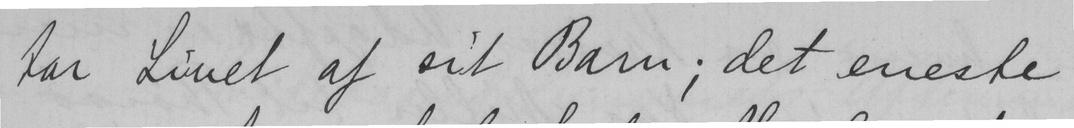tar Livet af sit Barn; det eneste
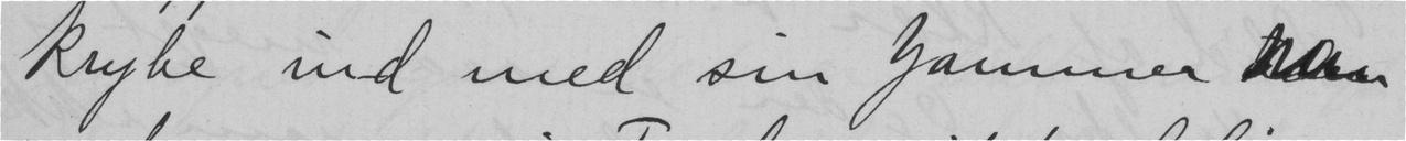krybe ind med sin Jammer
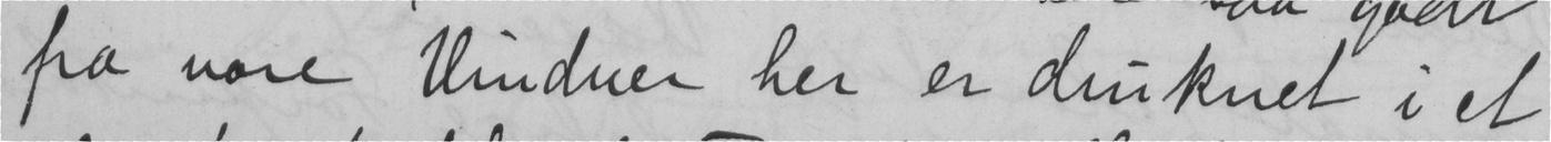fra vore vinduer her er druknet i et
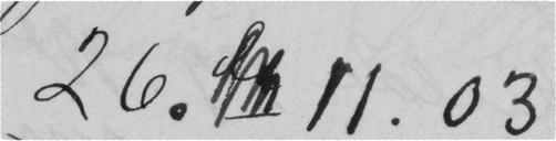26..11.03
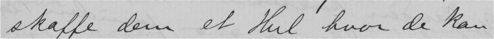skaffe dem et Hul hvor de kan
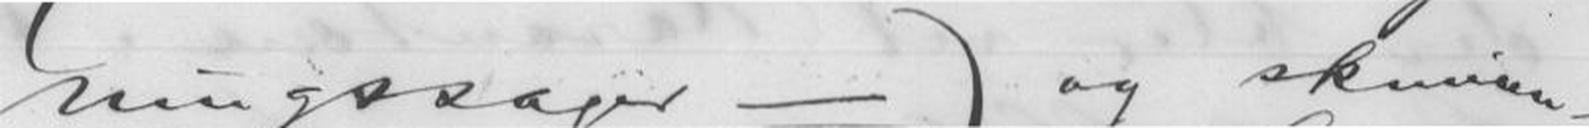ningssager -) og skinnen-
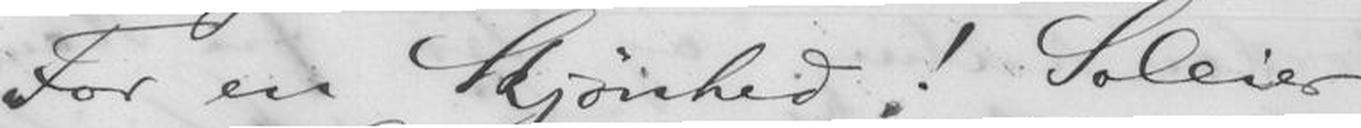For en Skjønhed! Soleier
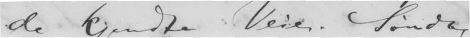de kjendte Veier. Søndag
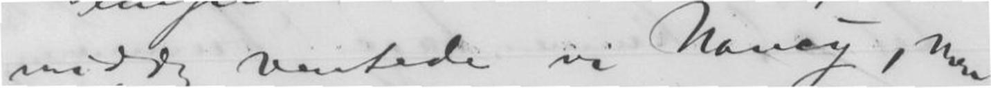middag ventede vi Nancy, men
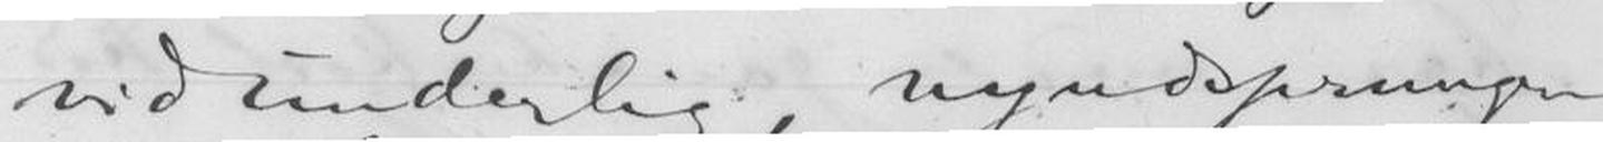vidunderlig, nyudsprungen
